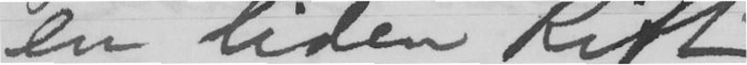en liden Rift -
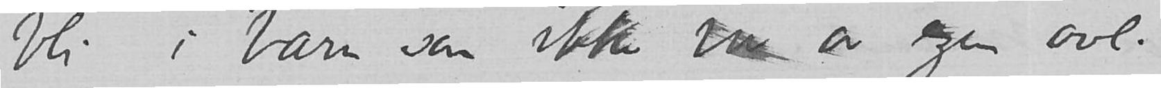bli i barn som ikke var av egen avl.
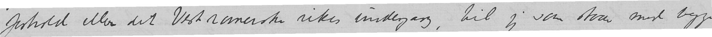eller det Vestromerske rikes undergang, til jeg som staar med begge
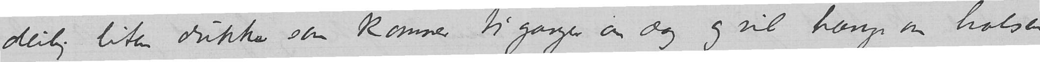deilig liten dukke som kommer ti ganger om dag og vil hænge om halsen
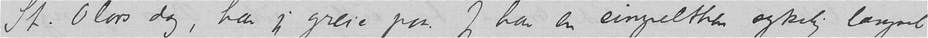St. Olavs dag, har jeg greie paa. Jeg har en simpelthen sykelig længsel
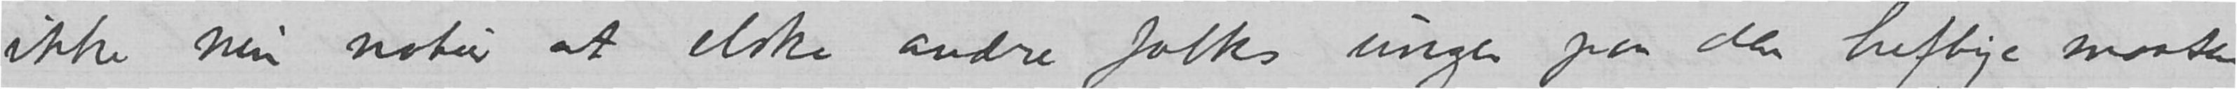ikke min natur at elske andre folks unger paa den heftige maaten
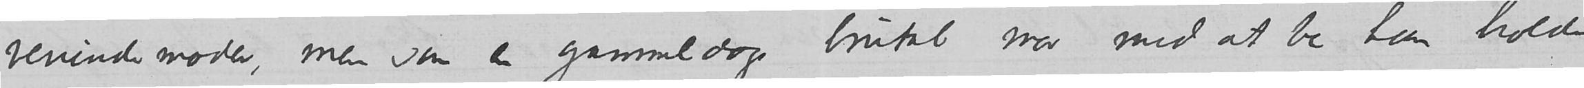venindemoder, men som en gammeldags brutal mor med at be ham holde
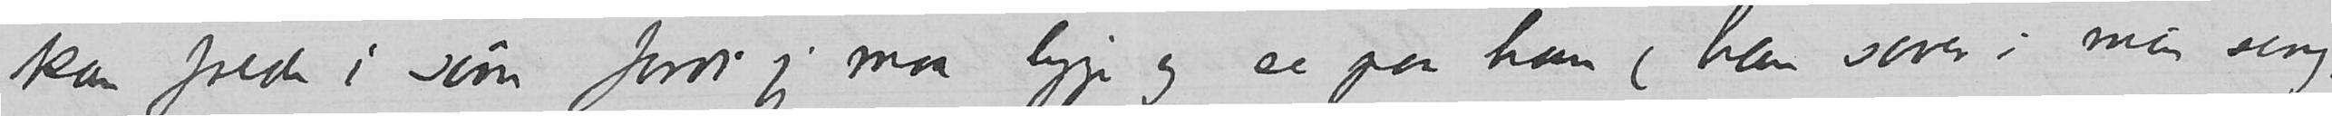kan falde i søvn fordi jeg maa ligge og se paa ham (han sover i min seng,
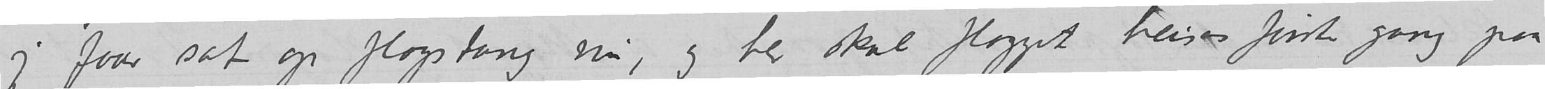jeg faar sat op flagstang nu, og her skal flagget heises første gang paa
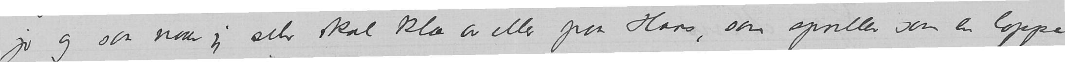med tillæg av avisreferat, – jo og saa naar jeg selv skal klæ av eller paa Hans, som spreller som en loppe
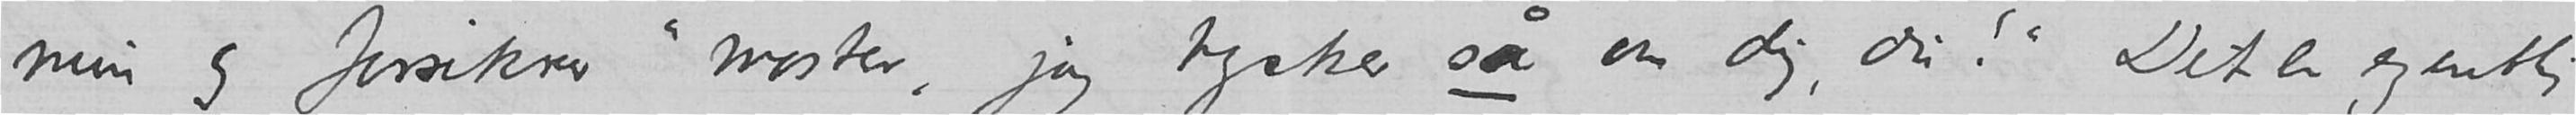min og forsikrer «moster, jag tycker så om dig, du!» Det er egentlig
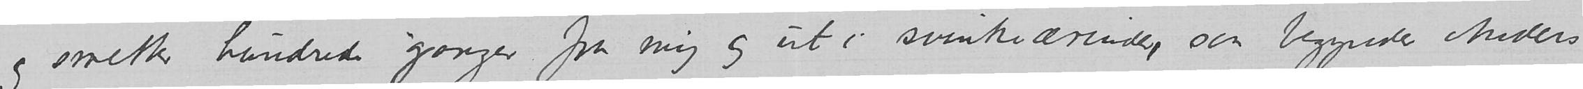og smetter hundrede ganger fra mig og ut i svinkeærinder, saa begynder Anders
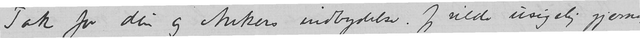Tak for din og Ankers indbydelse. Jeg vilde usigelig gjerne,
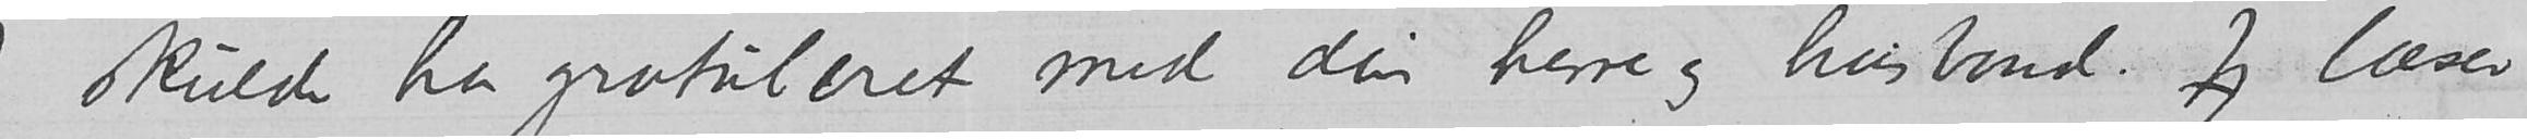Jeg skulde ha gratuleret med din herre og husbond. Jeg læser
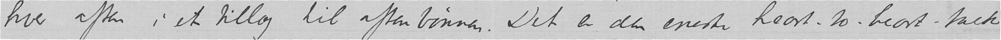hver aften i et tillæg til aftenbønnen. Det er den eneste heart-to-heart-talk
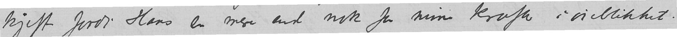kjeft fordi Hans er mere end nok for mine kræfter i øieblikket.
